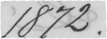1872.
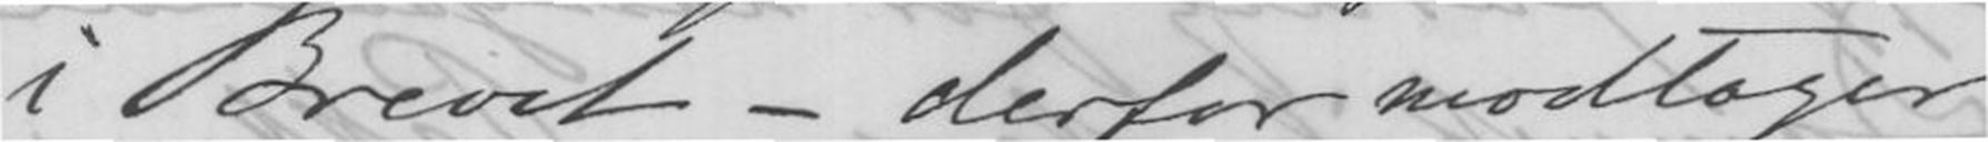i Brevet - derfor modtager
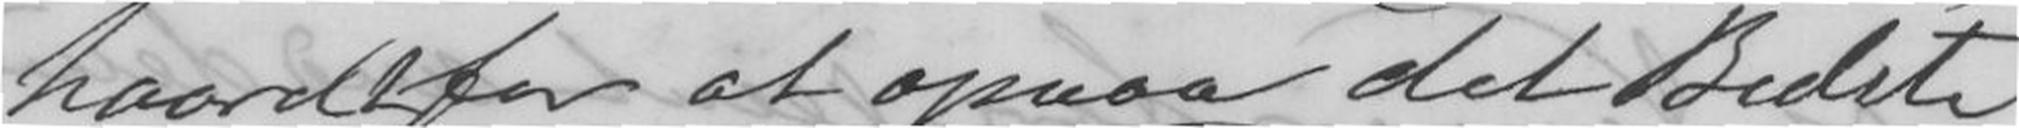haardt for at opnaa det Bedste
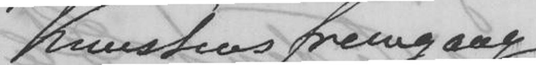nemligt til Kunstens fremgang
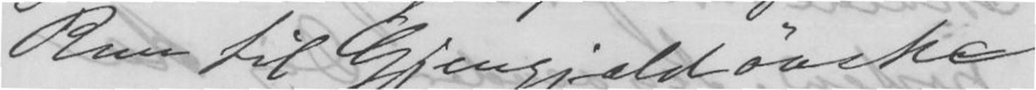kun til Gjengjæld ønske
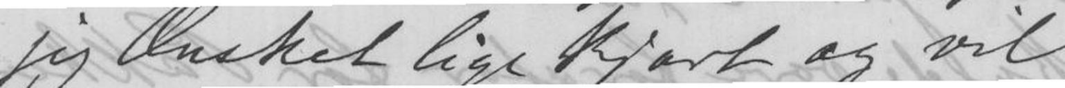jeg Ønsket lige kjært og vil
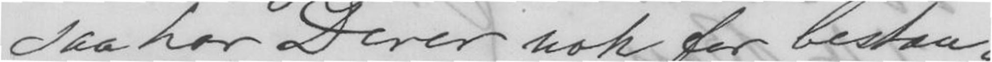saa har Derer nok for bestan-
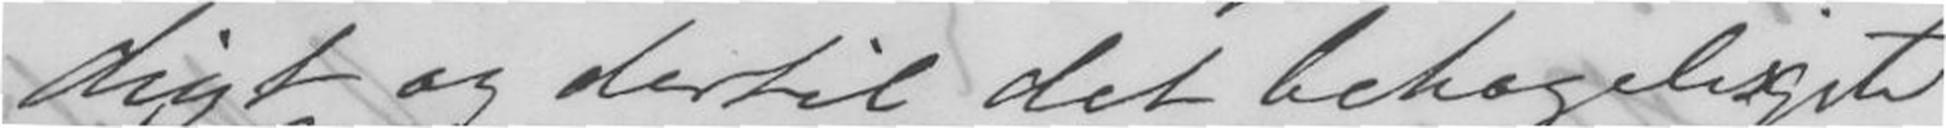digt og dertil det behageligste
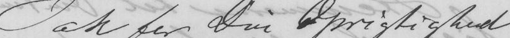Tak for Din Oprigtighed
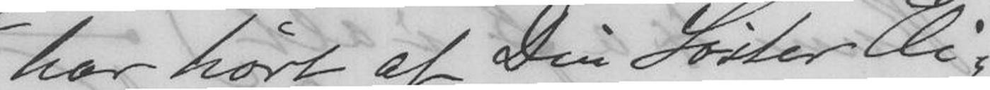Jeg har hørt af Din Søster Eli-
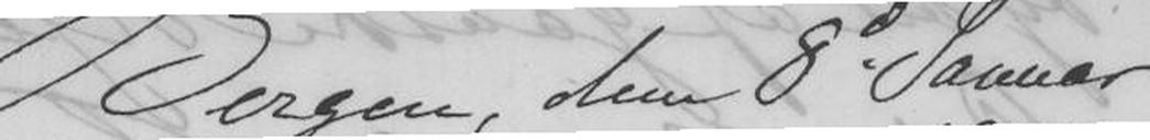Bergen, den 8d Januar
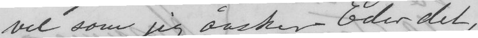vel som jeg ønsker Eder det,
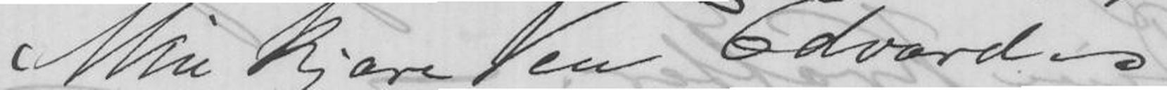Min kjære Ven Edvard.
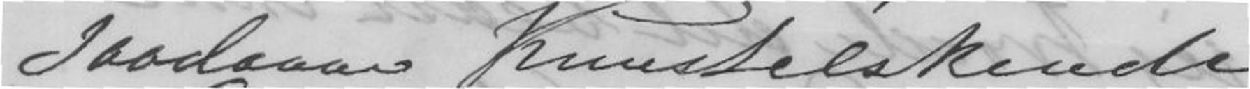saadanne Kunstelskende
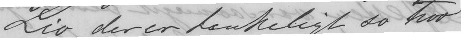Liv der er tænkeligt so tror
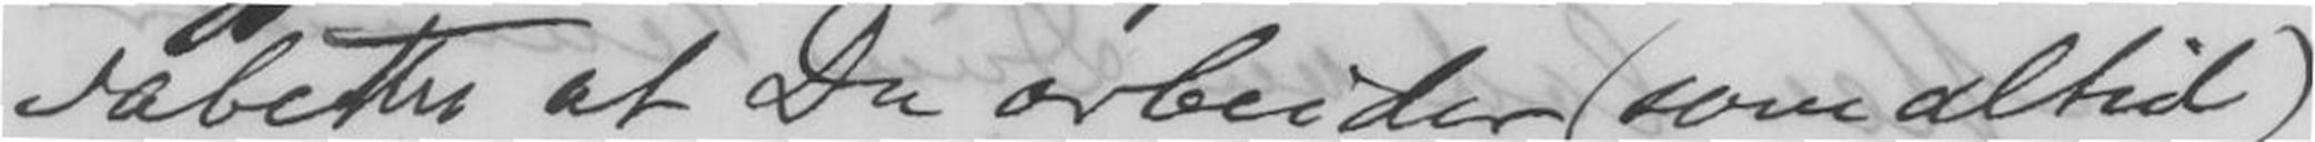sabeth at Du arbeider (som altid)
